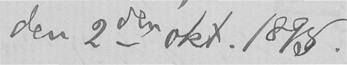den 2den okt. 1895.
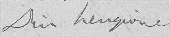Din hengivne
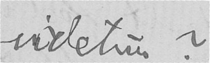videtur?
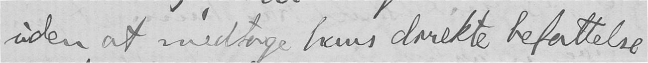uden at medtage hans direkte befattelse
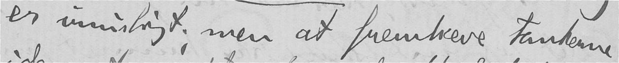er umuligt; men at fremhæve tankerne,
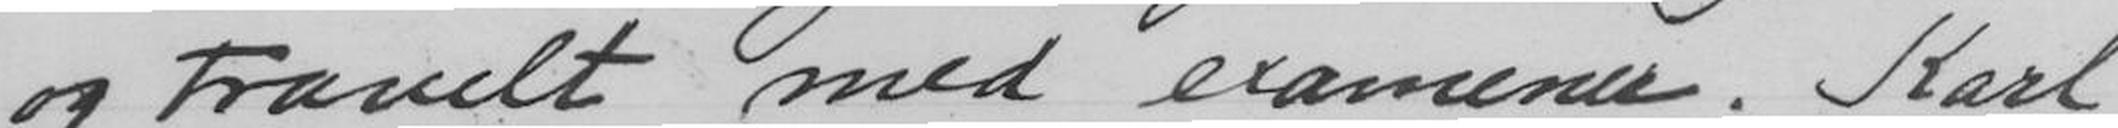og travelt med examener. Karl
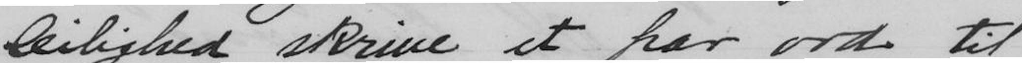leilighed skrive et par ord til
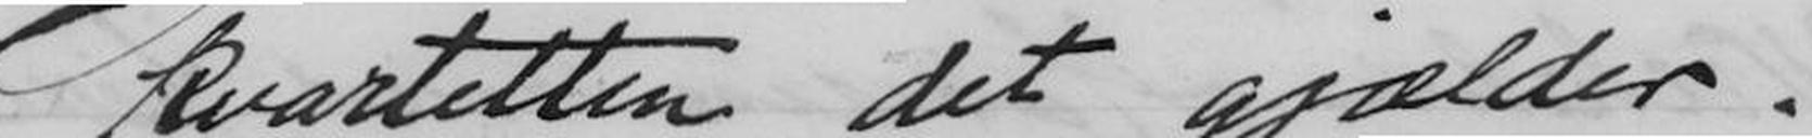kvartetten det gjælder.
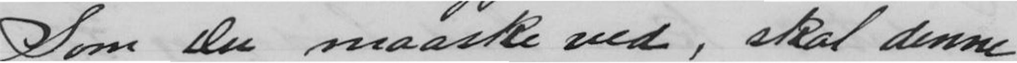Som Du maaske ved, skal denne
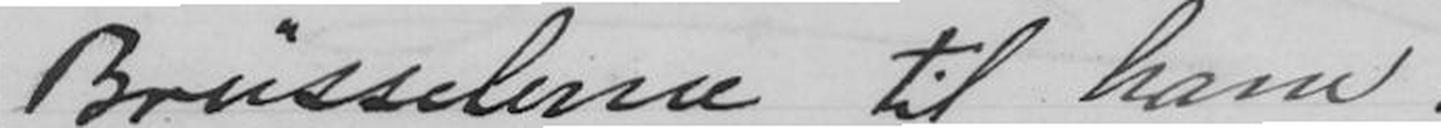Brüsselerne til ham.
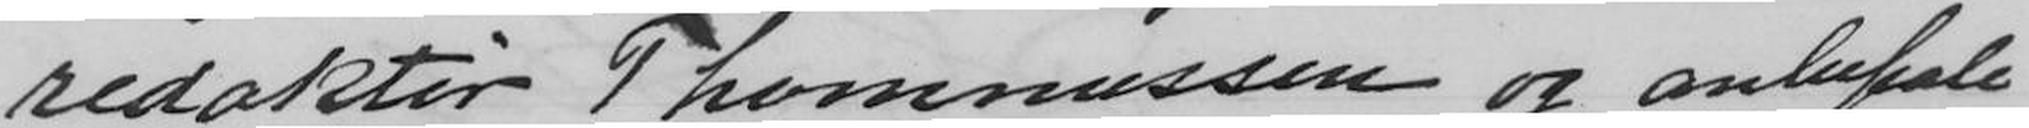redaktør Thommessen og anbefale
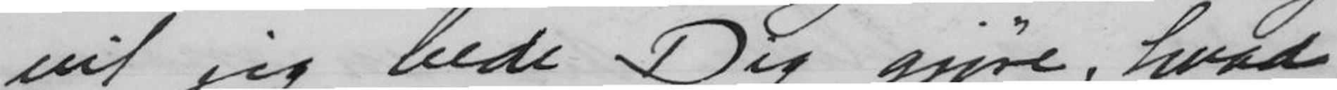vil jeg bede Dig gjøre, hvad
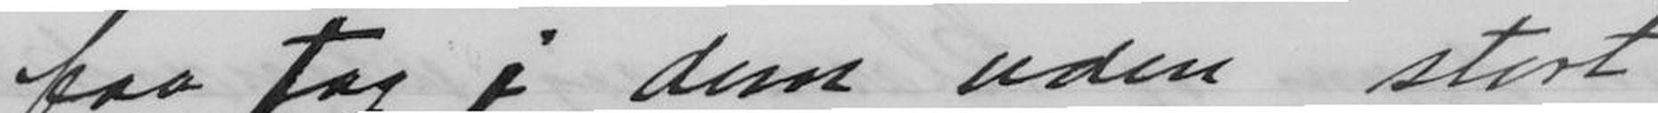faa tag i dem uden stort
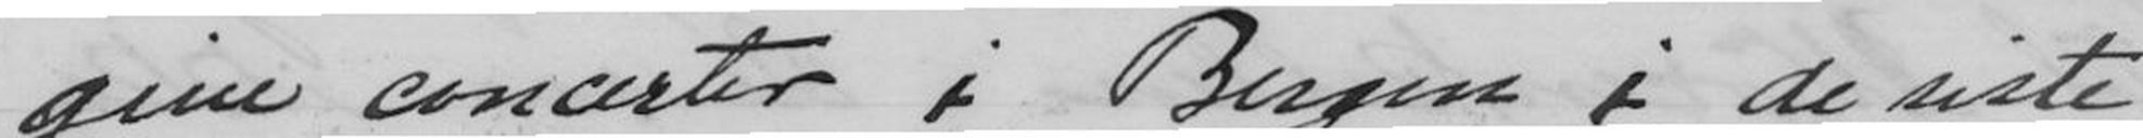give concerter i Bergen i de siste
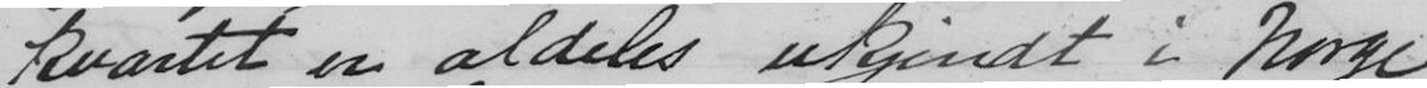kvartet er aldeles ukjendt i Norge
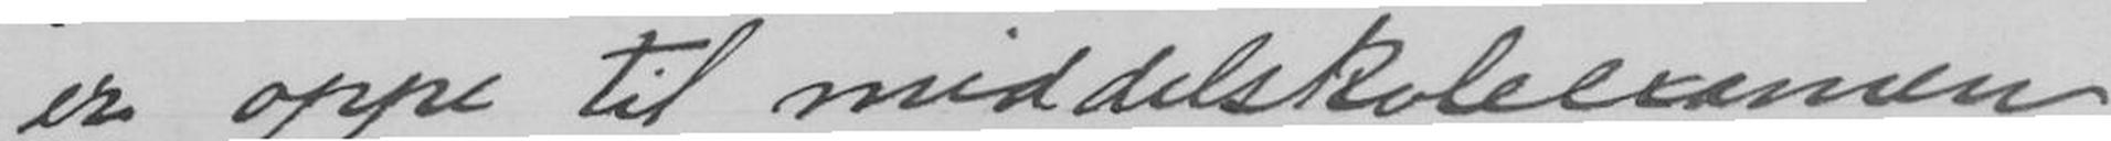er oppe til middelskoleexamen
